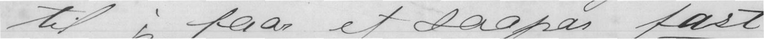til jeg faar et saapas fast
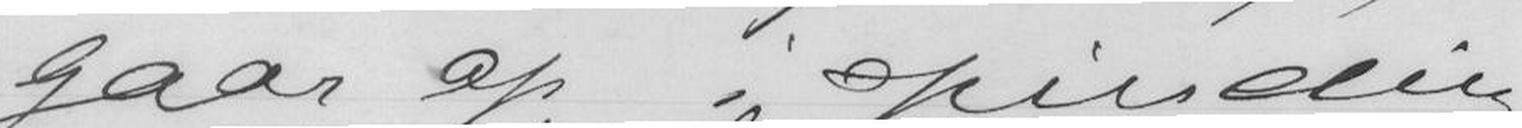gaar op i spinding.
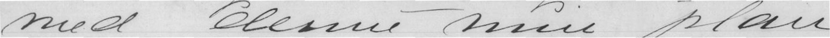med denne min plan
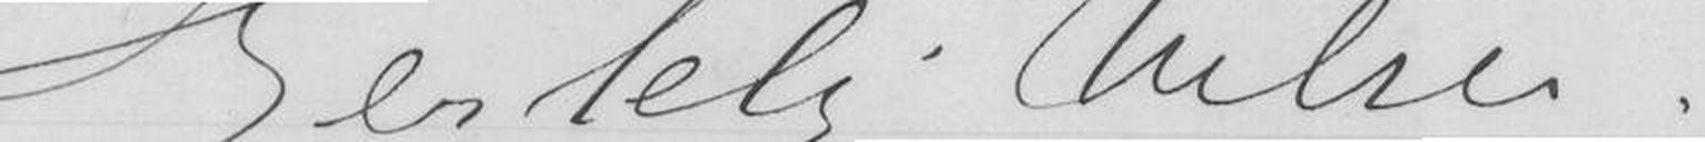Hjertelig hilsen.
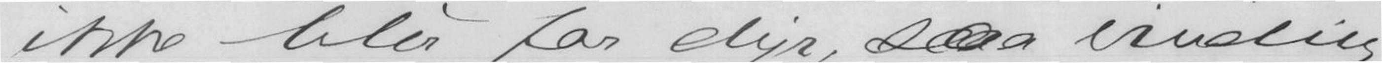ikke blir for dyr, saa vinding
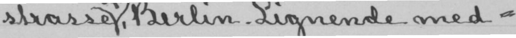strasse 4, Berlin. Lignende med-
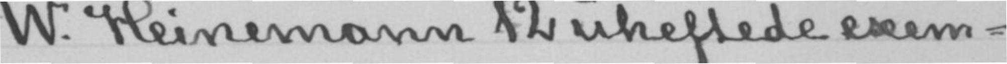W. Heinemann 12 uheftede exem-
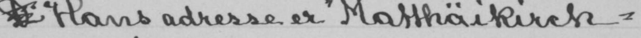D Hans adresse er Matthäikirch-
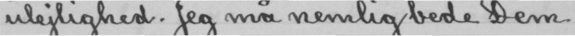ulejlighed. Jeg må nemlig bede Dem
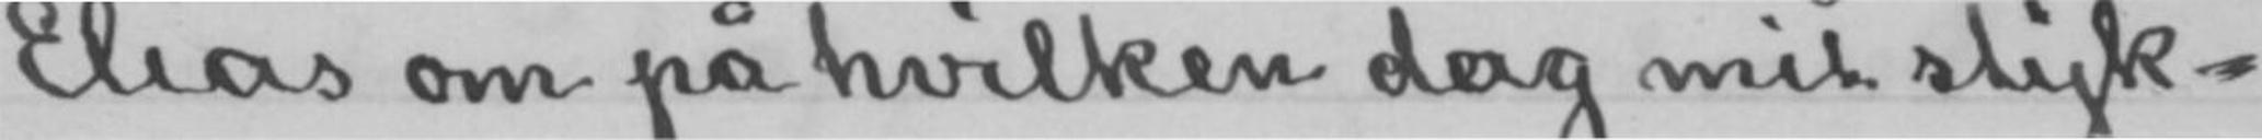Elias om på hvilken dag mit styk-
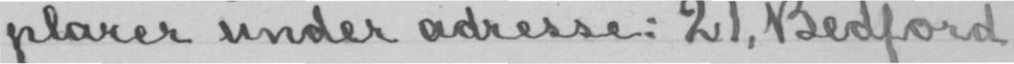plarer under adresse: 21, Bedford
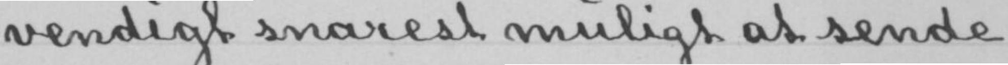vendigt snarest muligt at sende
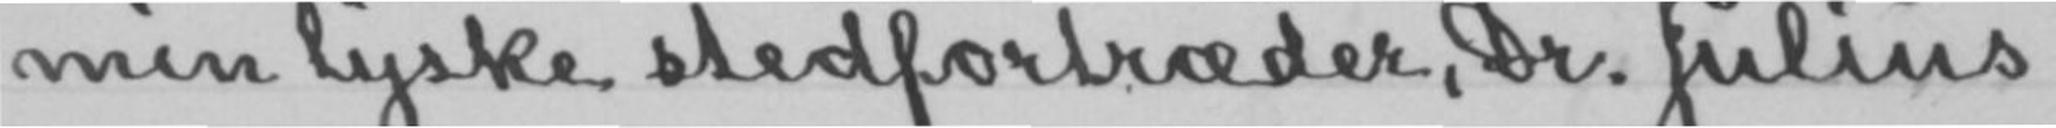min tyske stedfortræder, Dr. Julius
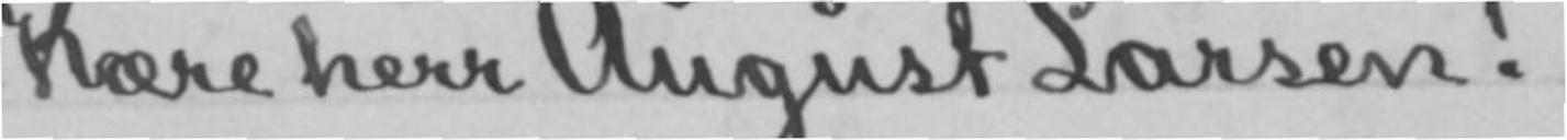Kære herr August Larsen!
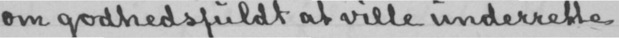om godhedsfuldt at ville underrette
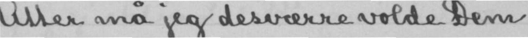Atter må jeg desværre volde Dem
